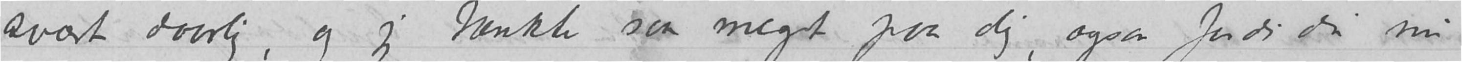svært daarlig, og jeg tenkte saa meget paa dig, også fordi du nu
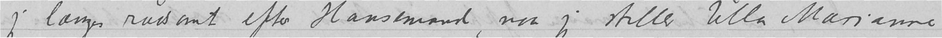jeg længes rædsomt efter Hansemand, naar jeg steller Ulla Marianne
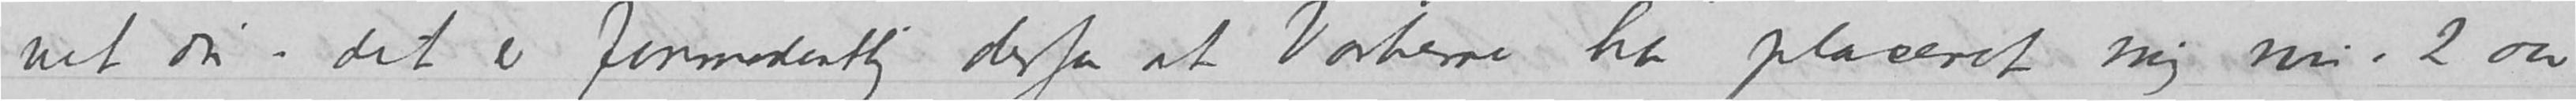vet du – det er formodentlig derfor at Vorherre har plaseret mig nu i 2 aar
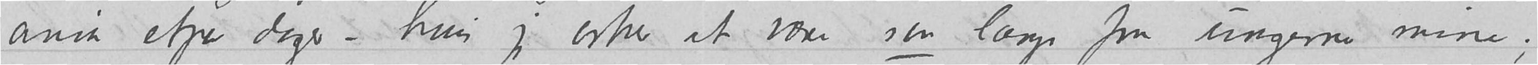ania etpar dager – hvis jeg orker at være saa længe fra ungerne mine;
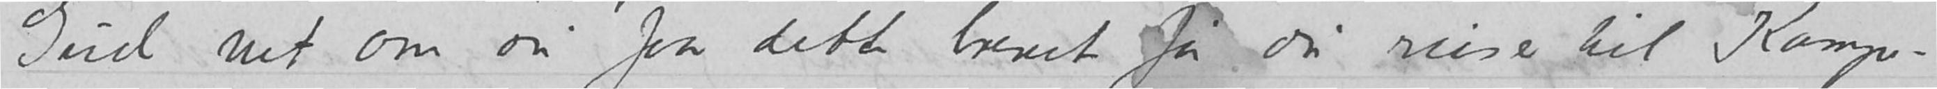Gud vet om du faar dette brevet før du reiser til Kampe-
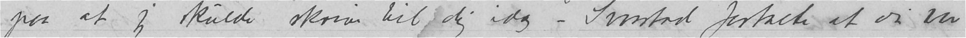paa at jeg skulde skrive til dig idag – Svarstad fortalte at du var
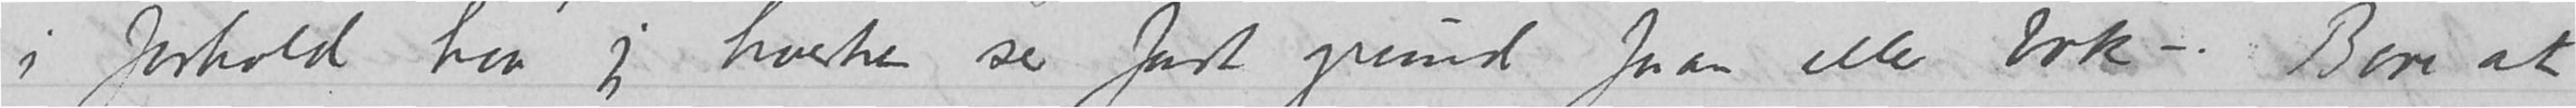i forhold hvor jeg hverken ser fast grund foran eller bak –.  Bare at
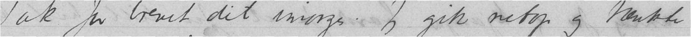Tak for brevet dit imorges.  Jeg gik netop og tænkte
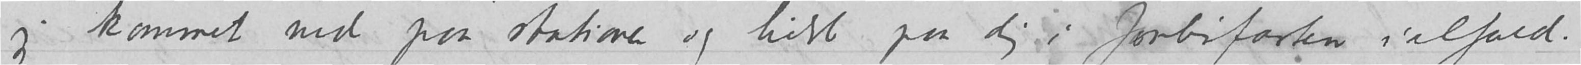jeg kommet ned paa stationen og hilst paa dig i forbifarten ialfald.
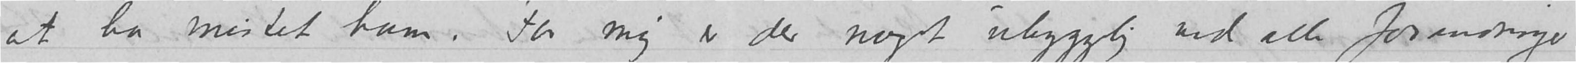at ha mistet ham.  For mig er der noget uhyggelig ved alle forandringer
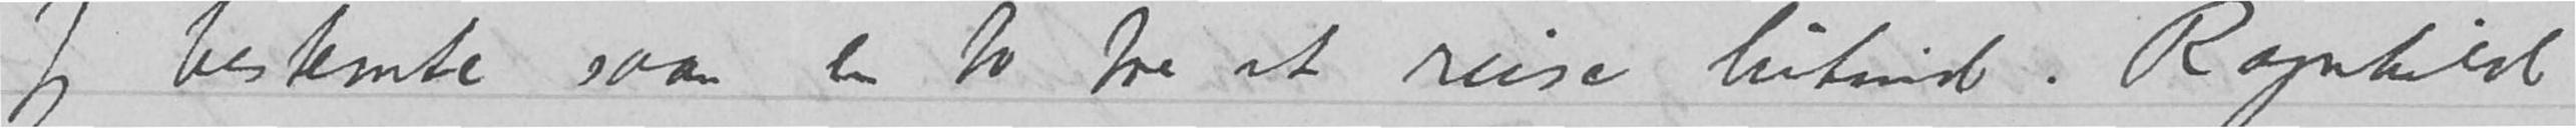Jeg bestemte saan en to tre at reise hitind.  Ragnhild
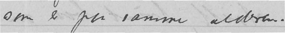som er paa samme alderen.
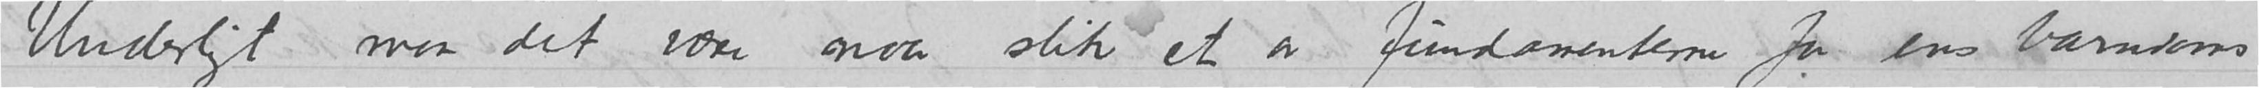Underligt maa det være naar slik et av fundamenterne fra ens barndoms
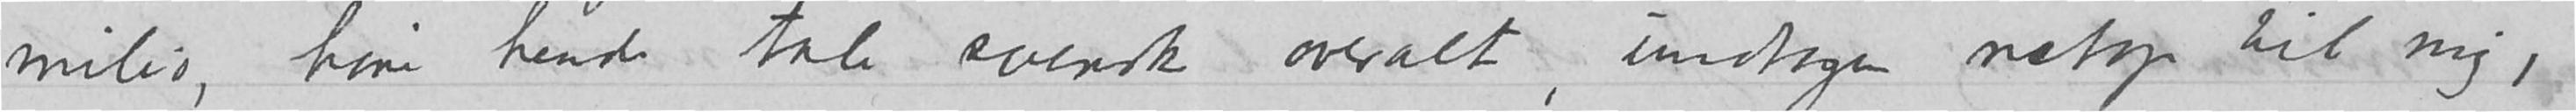miljø, høre hende tale svensk overalt, undtagen netop til mig,
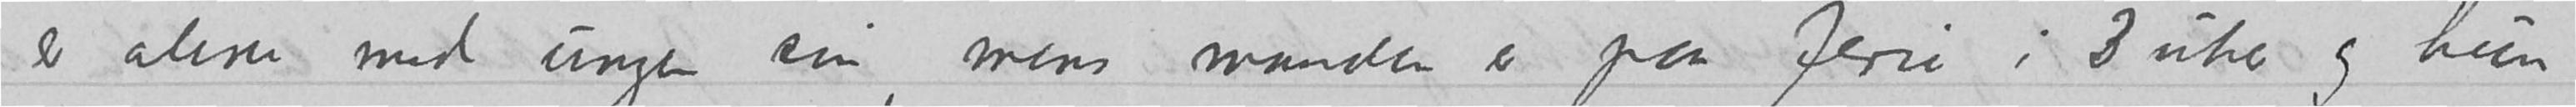er alene med ungen sin, mens manden er paa ferie i 3 uker og hun
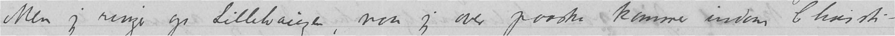Men jeg ringer op Lillehaugen, naar jeg over paaske kommer indom Christi-
